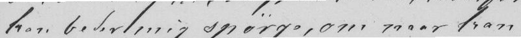han beder mig spørge, om naar han
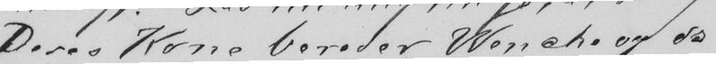Deres Kone bereder Wenche og os
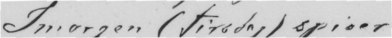Imorgen (tirsdag) spiser
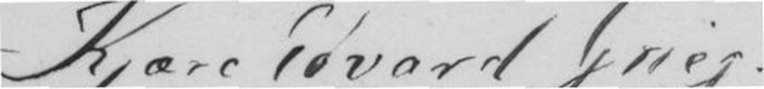Kjære Edvard Grieg!
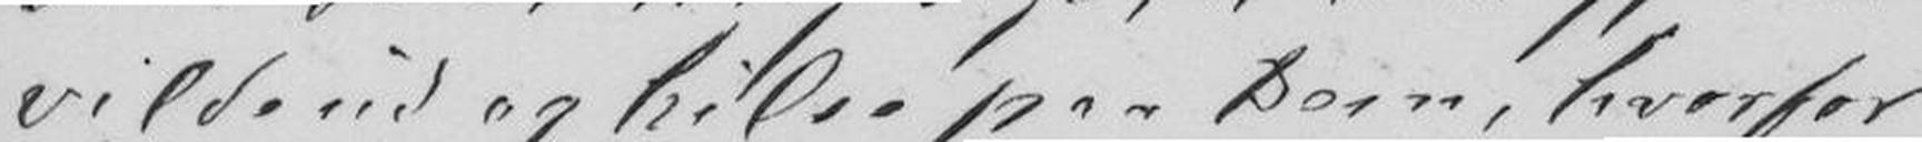vilde ud og hilse paa Dem, hvorfor
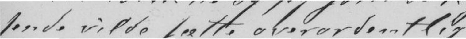sende vilde sætte overordentlig
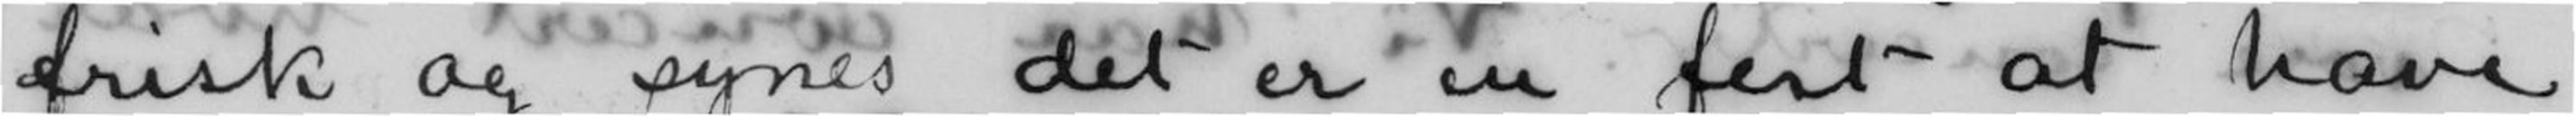frisk og synes det er en fest at have
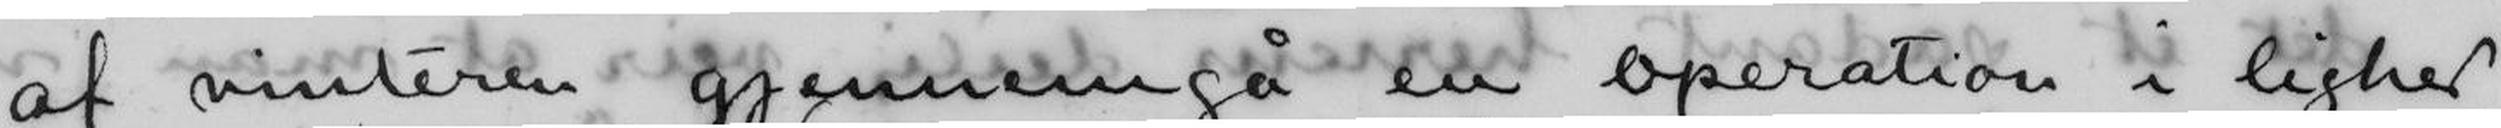af vinteren gjennemgå en operation i lighed
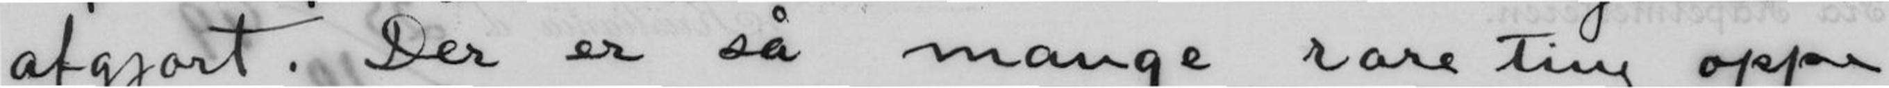afgjort. Der er så mange rare ting oppe
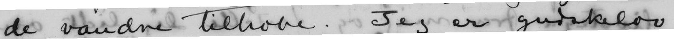de vandrer tilhobe. Jeg er gudskelov
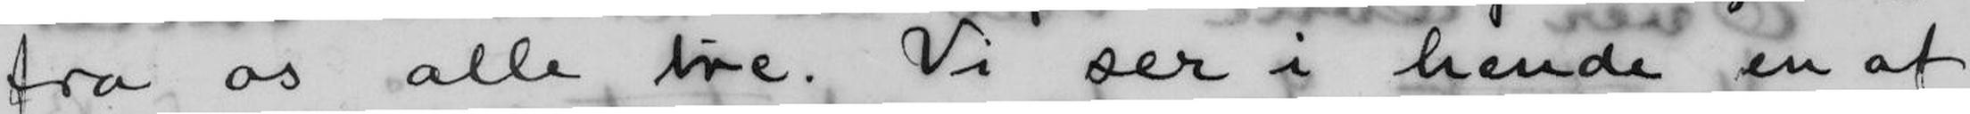fra os alle tre. Vi ser i hende en af
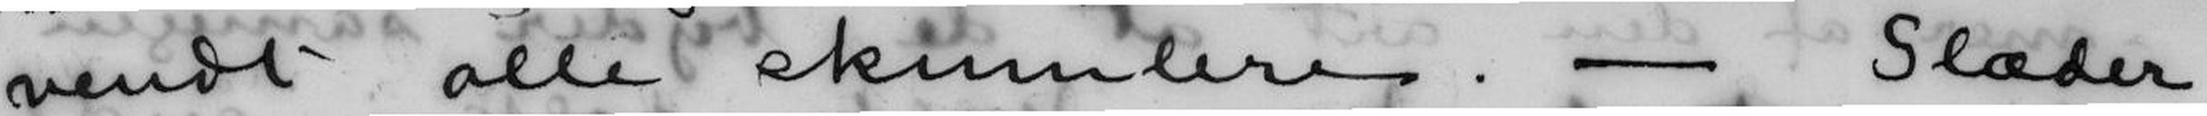vendt alle skumlere. -- Glæder
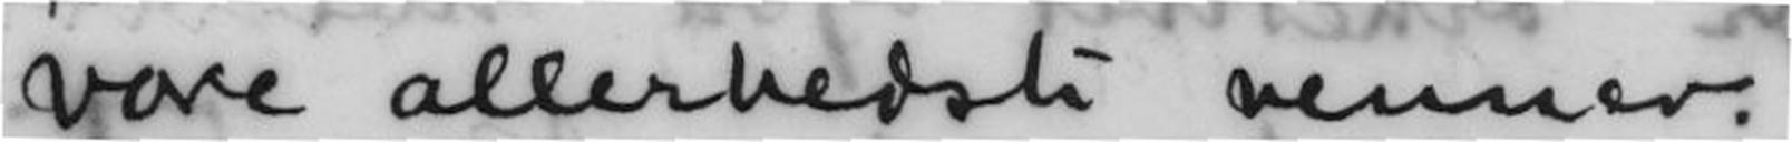vore allerbedste venner.
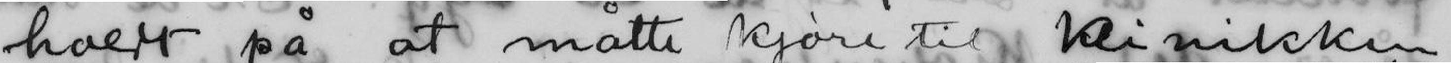holdt på at måtte kjøre til klinikken.
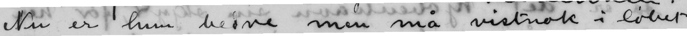Nu er hun bedre men må vistnok i løbet
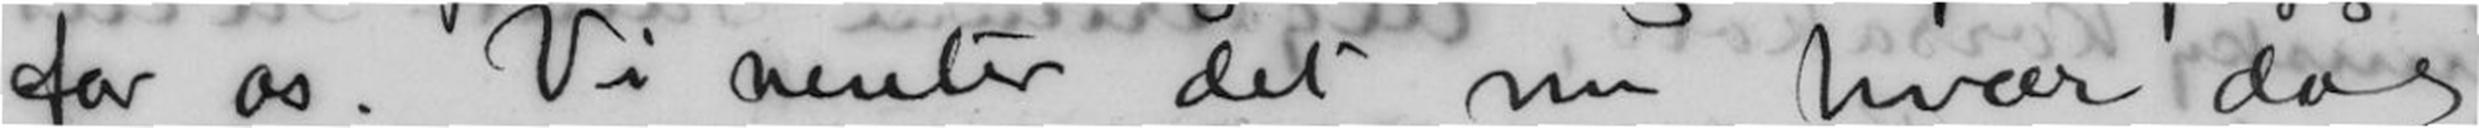for os. Vi venter det nu hvær dag.
